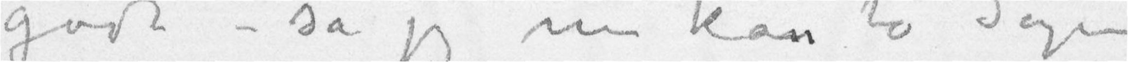godt – så jeg nu kan ta Sagen
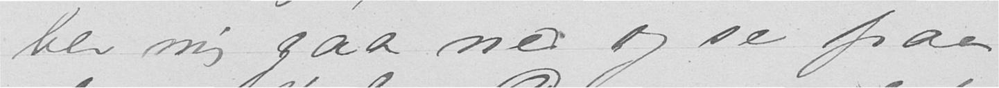ber mig gaa ned og se paa
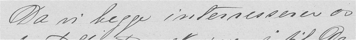Da vi begge interresserer os
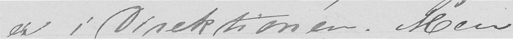er i Direktionen. Men
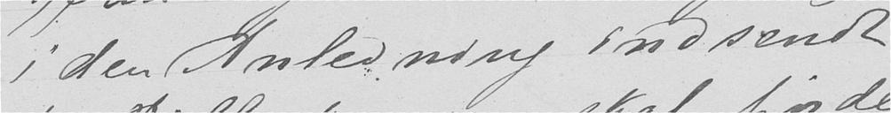i den Anledning indsendt
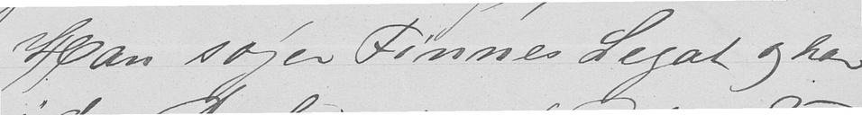Han søger Finnes Legat og har
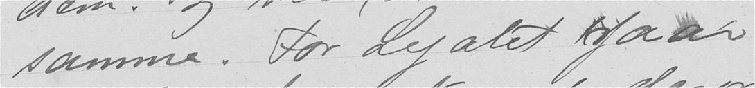samme. For Legatet hfaar
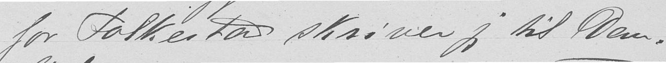for Folkestad skriver jeg til Dem.
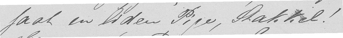faat en liden Pige, Stakkel!
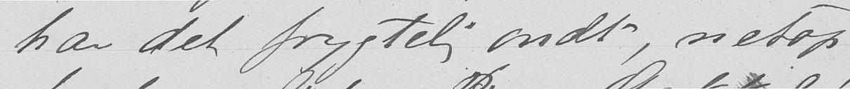har det frygtelig ondt, netop
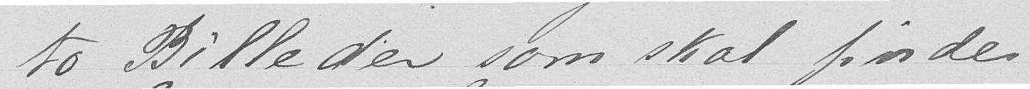to Billeder som skal findes
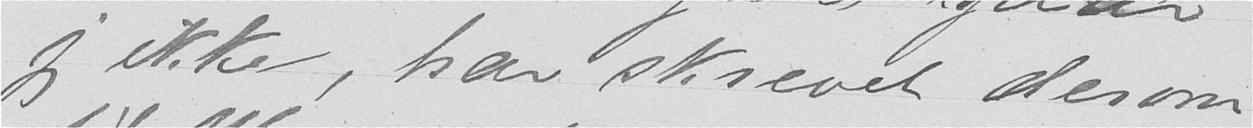jeg ikke, har skrevet derom
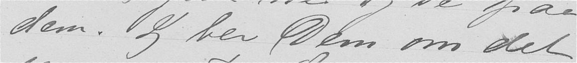dem. Jeg ber Dem om det
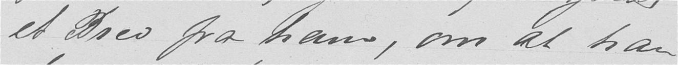et Brev fra ham, om at han
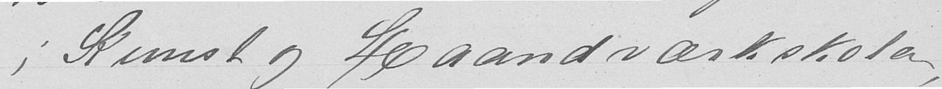i Kunst og Haandværkskolen,
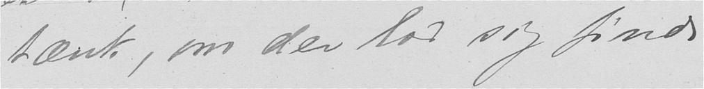tænk, om der lod sig finde
